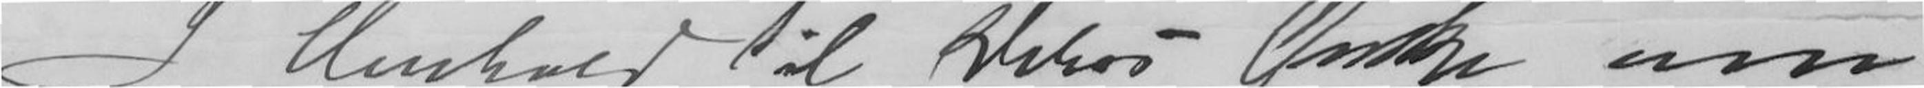I Henhold til Deres Ønske om
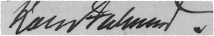Kom Formand.
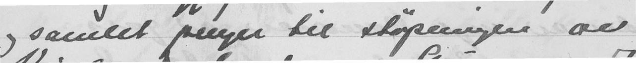og samlet penger til støpningen av
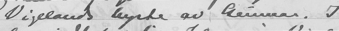Vigelands byste av Gunnar. I
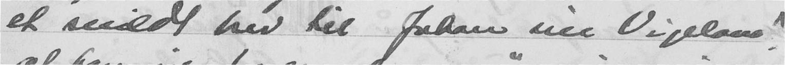et snildt brev til Johan sa Vigeland
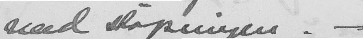med støpningen. -
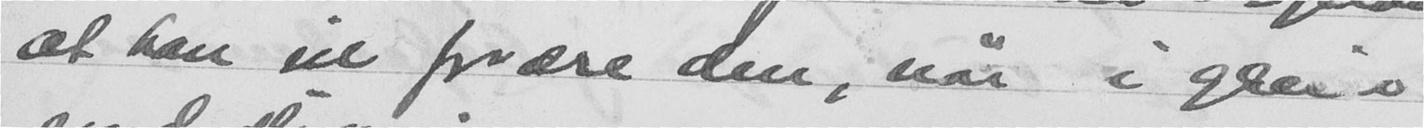at han vil forære den, nar i ganrs
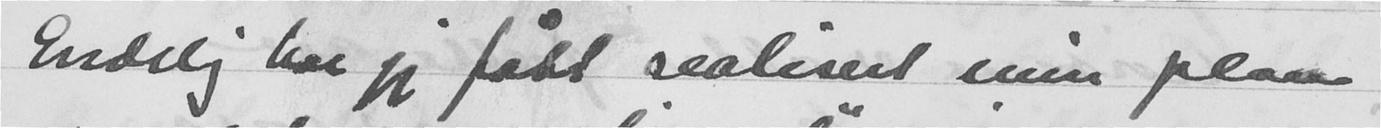Endelig har jeg fått realisert min plan
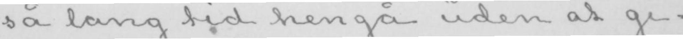så lang tid hengå uden at gi-
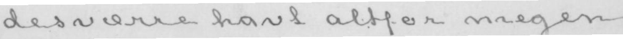desværre havt altfor megen
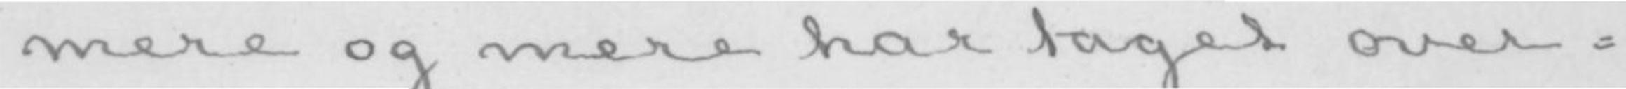mere og mere har taget over-
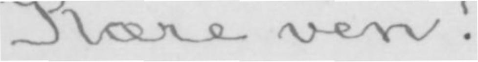Kære ven!
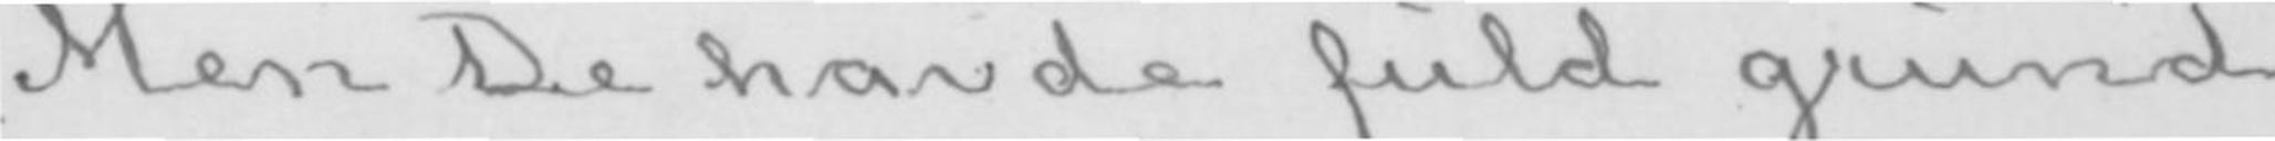Men De havde fuld grund
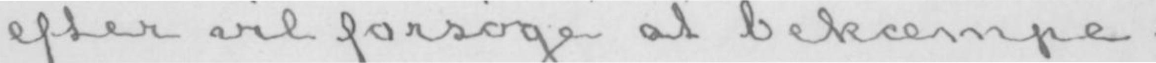efter vil forsøge at bekæmpe.
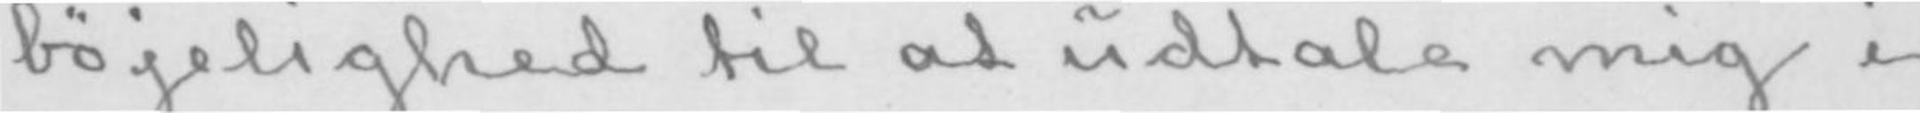bøjelighed til at udtale mig i
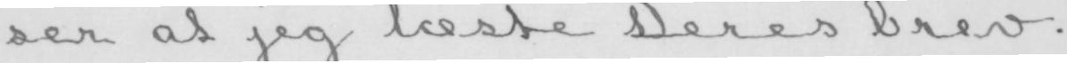ser at jeg læste Deres brev.
